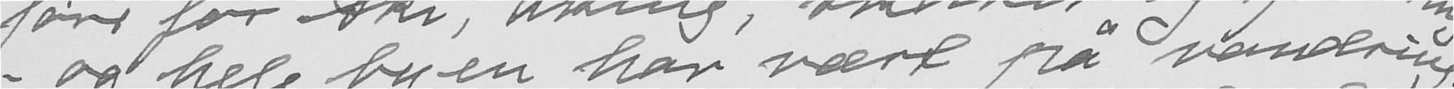- og hele byen har vært på vandring!
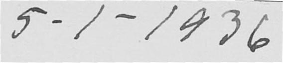5-1-1936
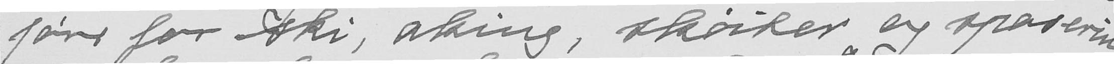føre for ski, aking, skøiter og spasering
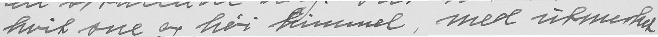hvit sne og høi himmel, med utmerket
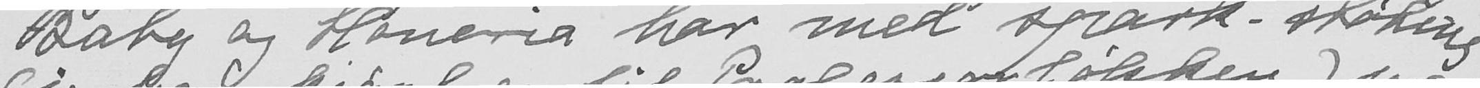Baby og Honoria har med spark-støting
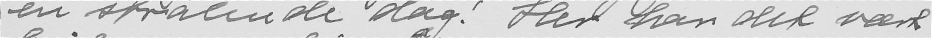en strålende dag! Her har det vært
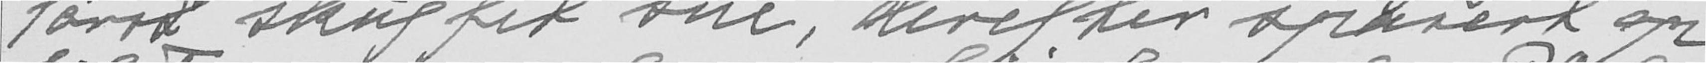først skuffet sne, derefter spasert op
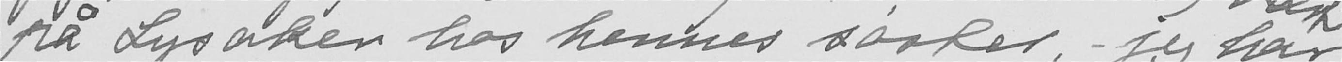på Lysaker hos hennes søster, - jeg har
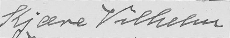Kjære Vilhelm
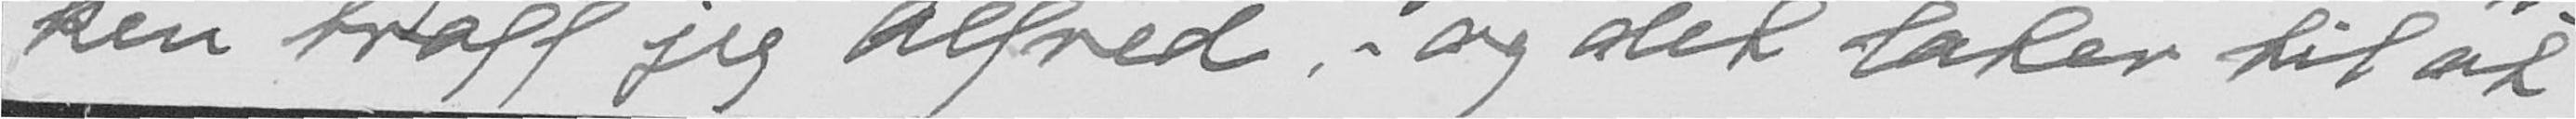ken traff jeg Alfred, - og det later til at
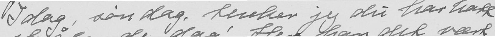I dag, søndag, tenker jeg du har hatt
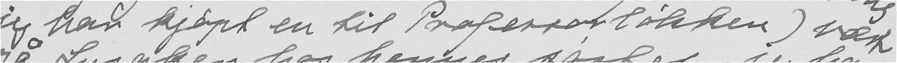(jeg har kjøpt en til Professorløkken) vært
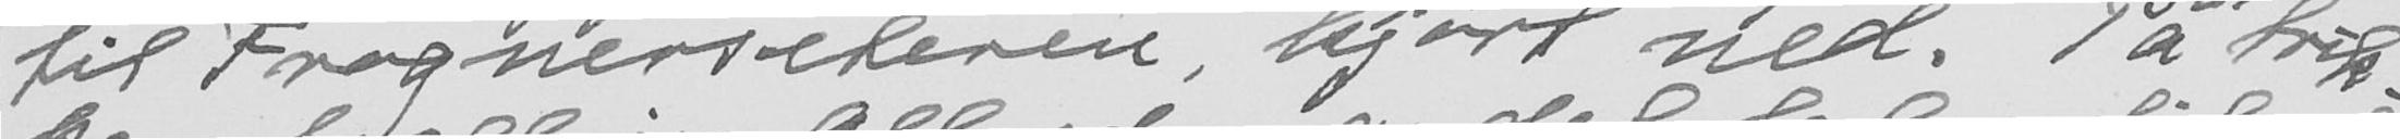til Frognerseteren, kjørt ned. På trik-
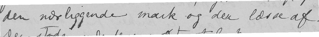den nærliggende mark og der læsse af.
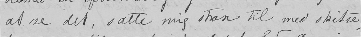at se det, satte mig strax til med skitse-
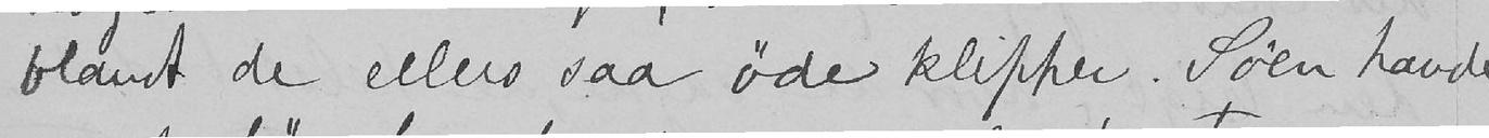blandt de ellers saa øde klipper. Søen havde
